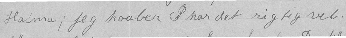Halma; Jeg haaber I har det rigtig vel.
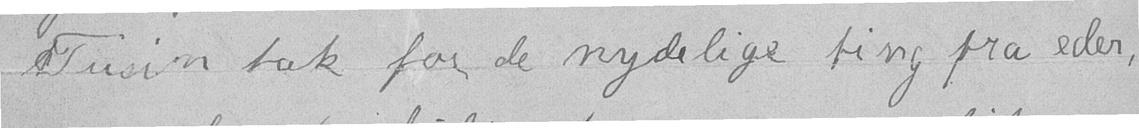Tusin tak for de nydelige ting fra eder,
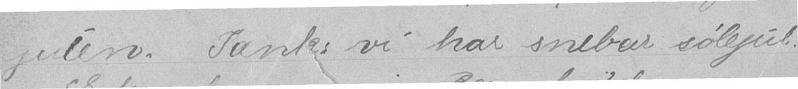julen. Tænk: vi har snebar sølejul.
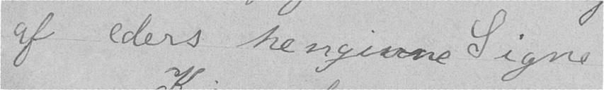af eders hengivne Signe
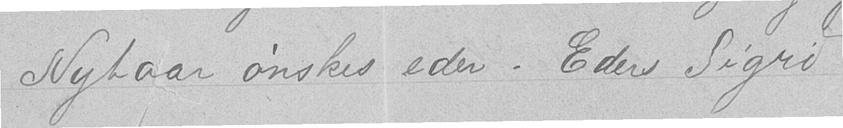Nytaar ønskes eder. Eders Sigrid
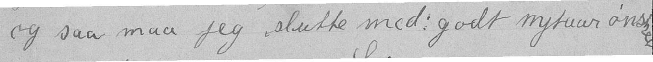og saa maa jeg slutte med: godt nytaar ønskes
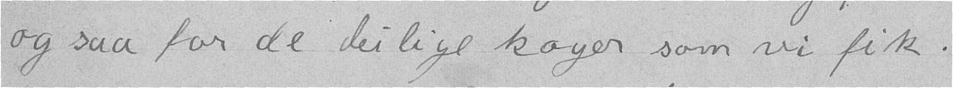og saa for de deilige kager som vi fik.
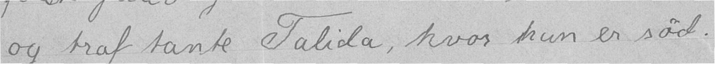og traf tante Talida, hvor hun er sød.
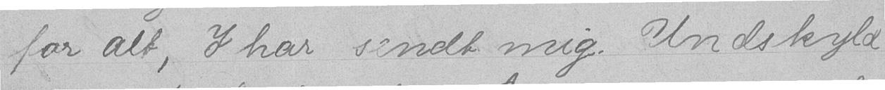for alt, I har sendt mig. Undskyld
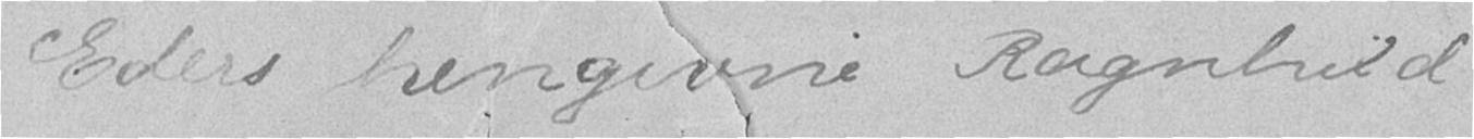Eders hengivne Ragnhild
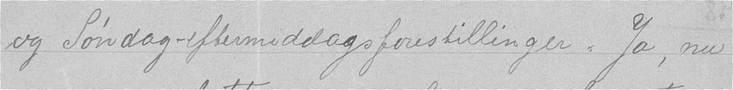og Søndag-eftermiddagsforestillinger. Ja, nu
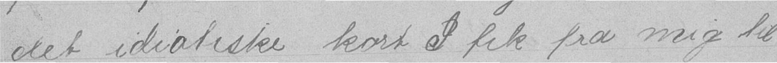det idiotiske kort I fik fra mig til
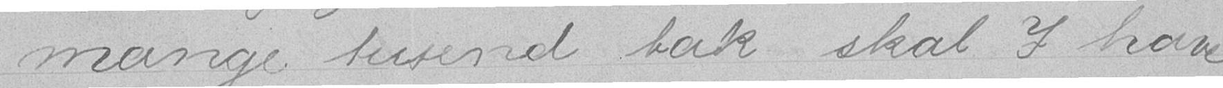mange tusend tak skal I have
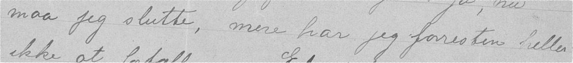maa jeg slutte, mere har jeg forresten heller
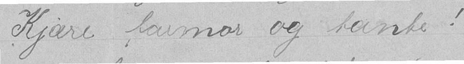Kjære farmor og tante!
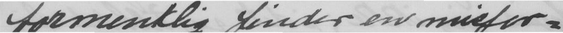formentlig finder en misfor-
